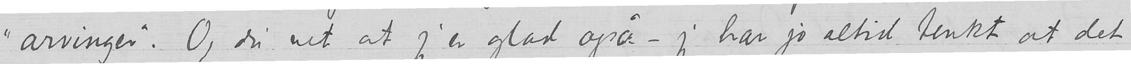"arvinger". Og du vet at jeg er glad også – jeg har jo altid tenkt at det
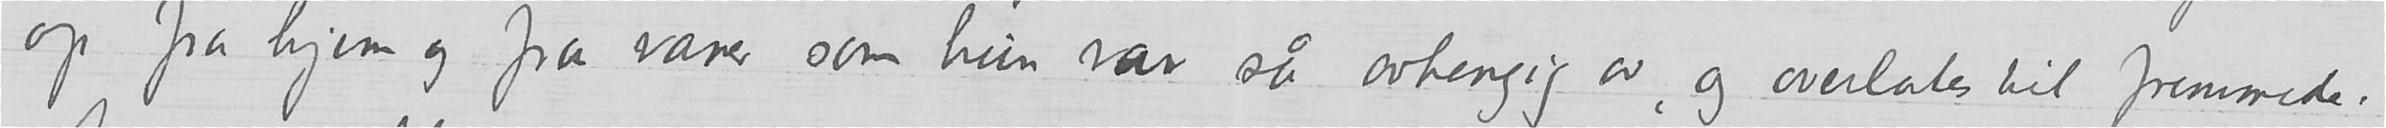op fra hjem og fra vaner som hun var si avhengig av, og overlates til fremmede.
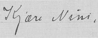Kjære Nini,
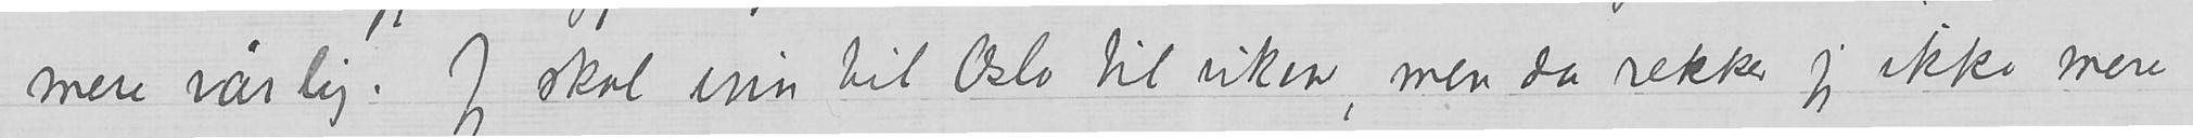mere vårlig. Jeg skal inn til Oslo til uken, men da rekker jeg ikke mere
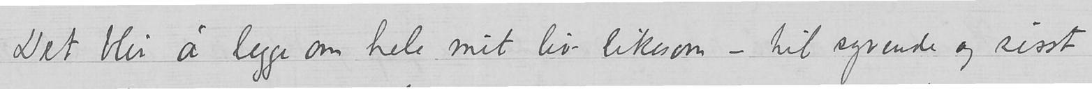Det blir å legge om hele mit liv likesom – til syvende og sisst
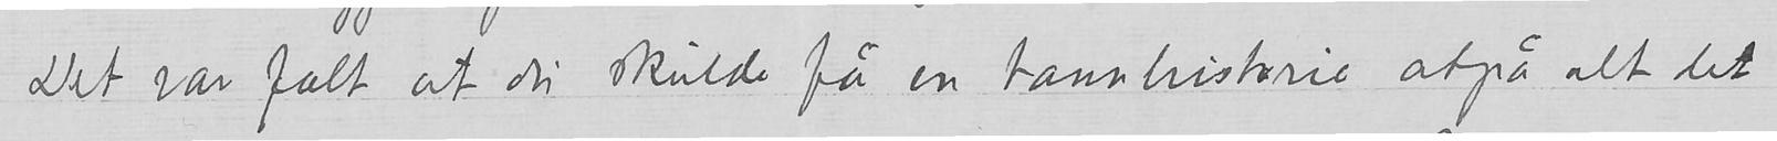Det var fælt at du skulde få en tannhistorie atpå alt det
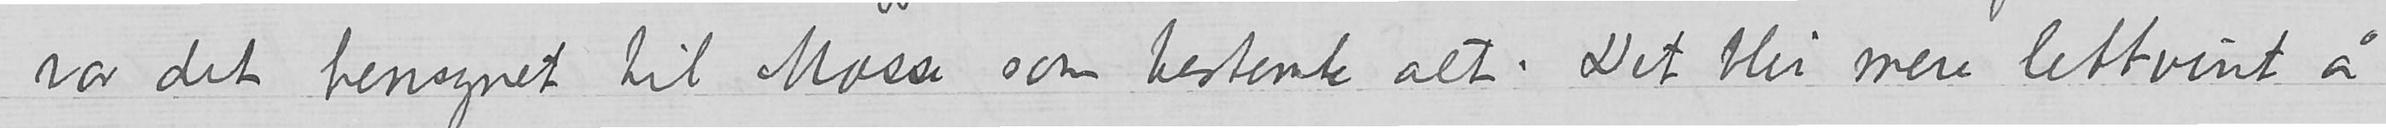var det hensynet til Mosse som bestemte alt. Det blir mere lettvint å
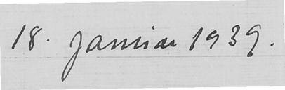18. januar 1939.
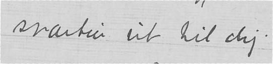svartur ut til dig.
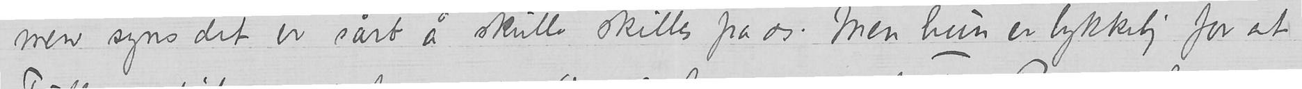men syns det er sårt å skulle skilles fra os. Men hun er lykkelig for at
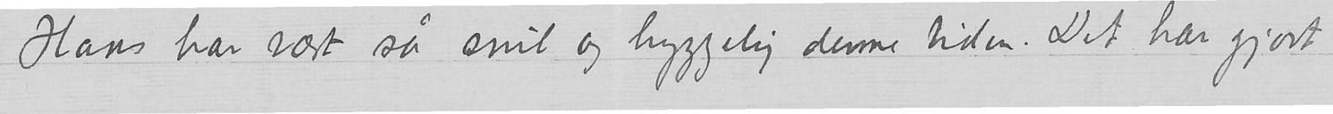Hans har vært så snil og hyggelig denne tiden. Det har gjort
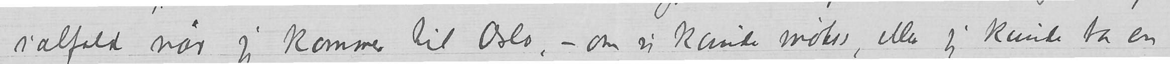ialfald når jeg kommer til Oslo, – om vi kunde møte, eller jeg kunde ta en
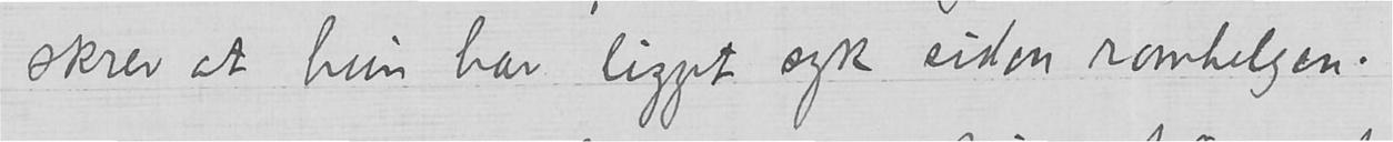skrev at hun har ligget syk siden romhelgen.
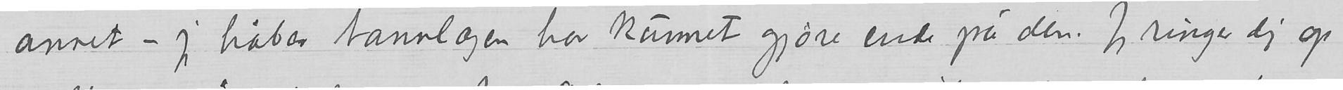annet – jeg håber tannlægen har kunnet gjøre ende på den. Jeg ringer dig op
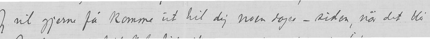Jeg vil gjerne få komme ut til dig noen dager – siden, når det blir
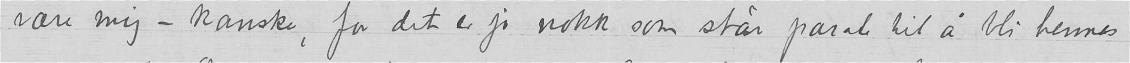være mig – kanske, for det er jo nokk som står parate til å bli hennes
